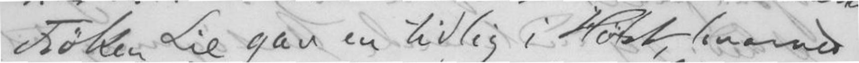Frøken Lie gav en tidlig i Høst, hvorved
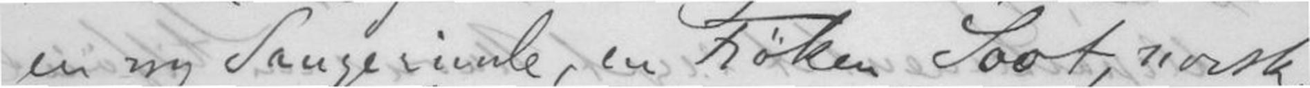en ny Sangerinde, en Frøken Soot, norsk,
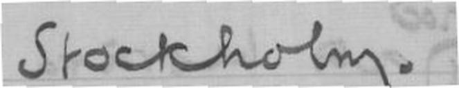Stockholm.
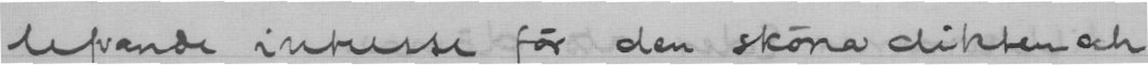lefvande intresse för den sköna dikten och
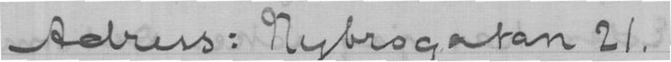Adress: Nybrogatan 21.
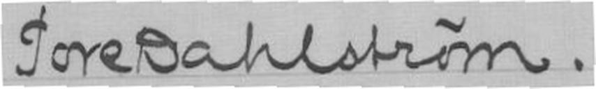Tore Dahlström
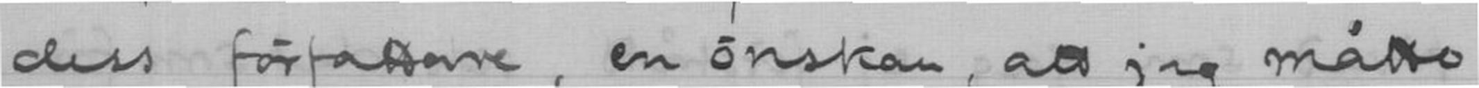dess författare, en önskan att jag måtte
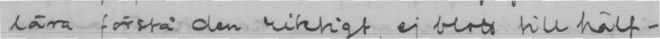lära förstå den riktigt, ej blott till hälf-
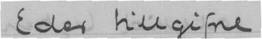Eder tillgifne
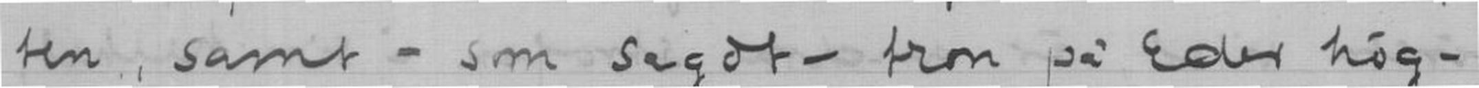ten, samt - som sagdt - tron på Eder hög-
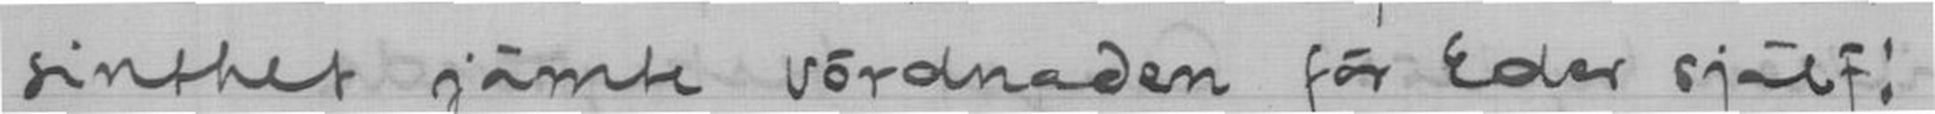sinthet jämte vördnaden för Eder själf!
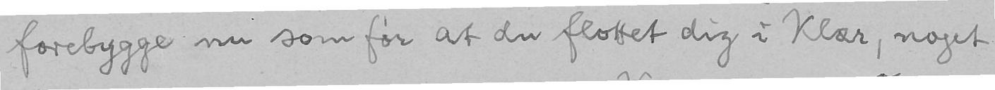forebygge nu som før at du flottet dig i Klær, noget
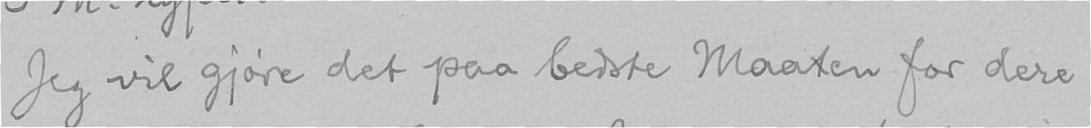Jeg vil gjøre det paa bedste Maaten for dere
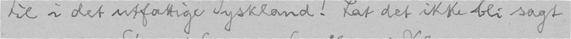til i det utfattige Tyskland! Lat det ikke bli sagt
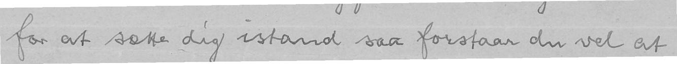for at sætte dig istand saa forstaar du vel at
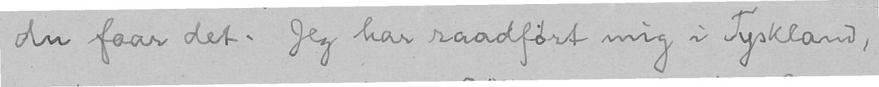du faar det. Jeg har raadført mig i Tyskland,
